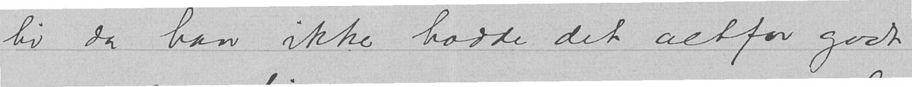liv da han ikke hadde det altfor godt
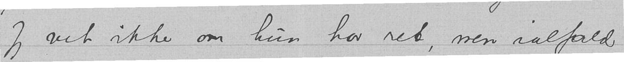Jeg vet ikke om hun har ret, men ialfald
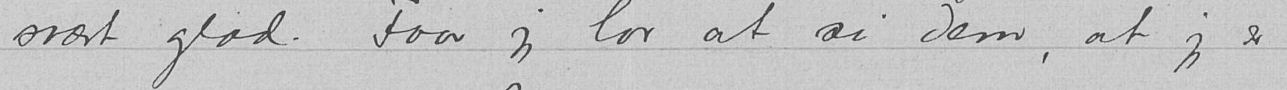svært glad. Faar jeg lov at si Dem, at jeg er
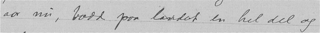aar nu, bodd paa landet en hel del og
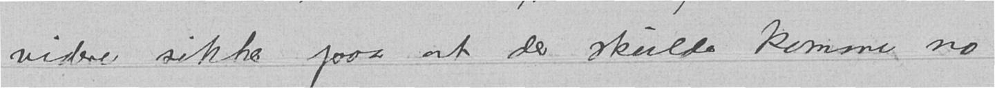videre sikker paa at der skulde komme no
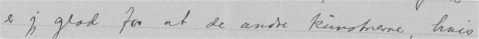er jeg glad for at de andre kunstnerne, hvis
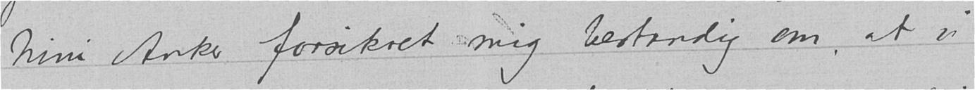Nini Anker forsikret mig bestandig om, at vi
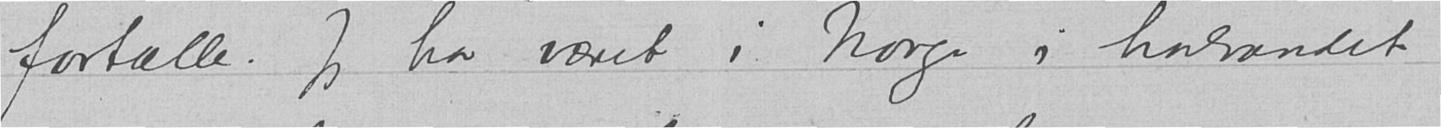fortælle. Jeg har været i Norge i halvandet
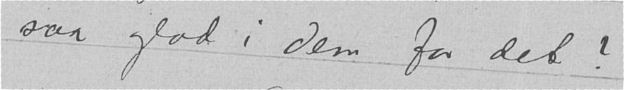saa glad i Dem for det?
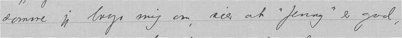domme jeg bryr mig om, sier at "Jenny» er god,
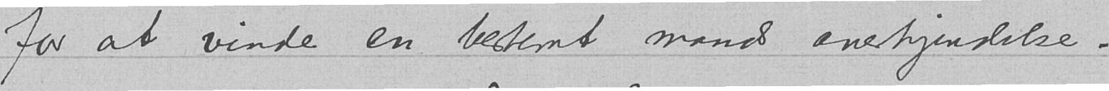for at vinde en bestemt mands anerkjendelse -
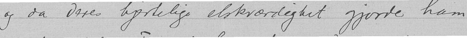og da Deres hjertelige elskværdighet gjorde ham
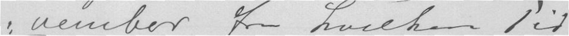vember for hvilken Tid
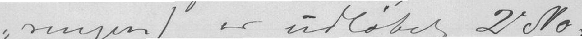ringen) er udløbet 2de No-
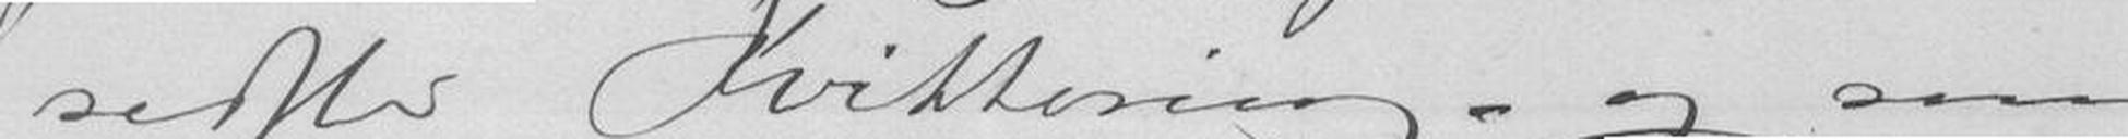sidste Kvittering - og som
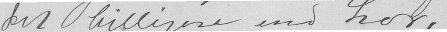det billigere end her, -
